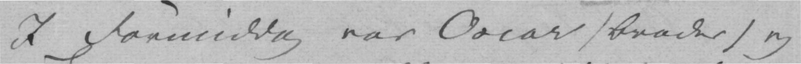I Formiddag var Oscar (Broder) og
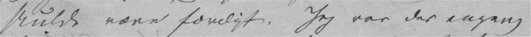skulde være færdigt. Jeg var der engang
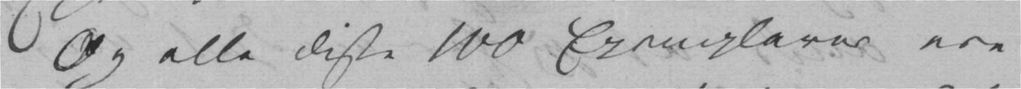Og alle disse 100 Exemplarer ere
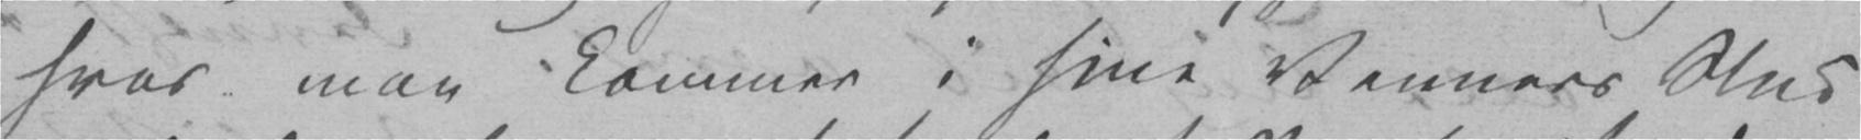hvor man kommer i sine Venners Stue
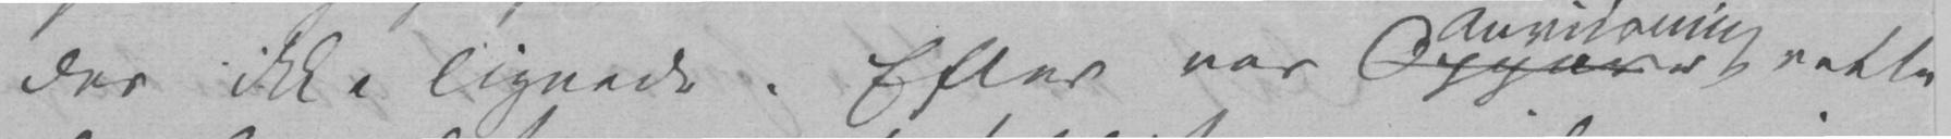der ikke lignede. Efter vor Opgave rette
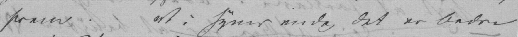frem. Vi synes endog det er bedre
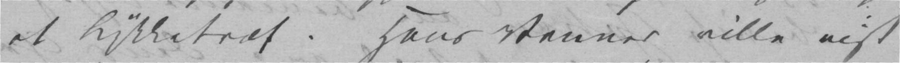et Lykketræf. Hans Venner ville vist
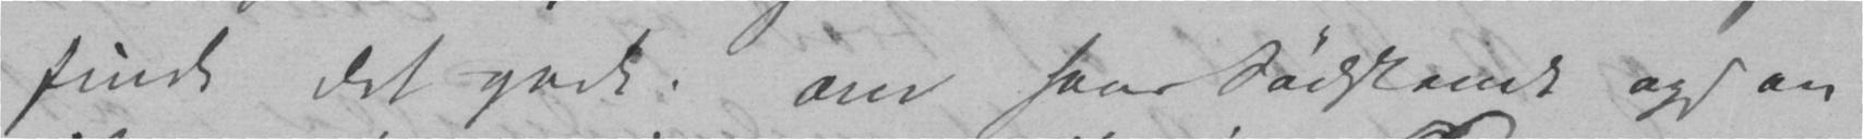finde det godt. om hans Sødskende ogsaa
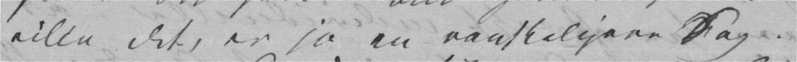ville det, er jo en vanskeligere Sag.
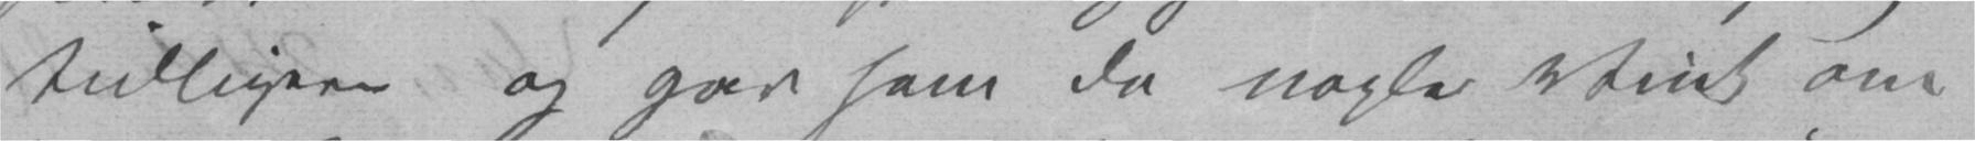tidligere og gav ham da nogle Vink om
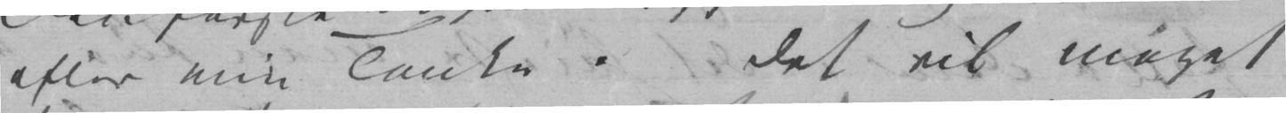efter min Tanke. Det vil meget
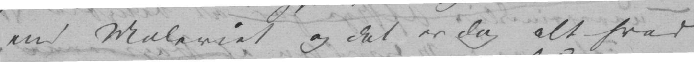end Maleriet og det er dog alt hvad
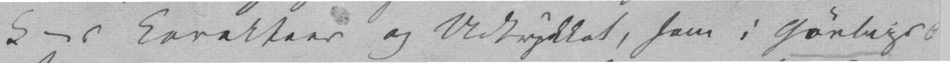C-s Carakteer og Udtrykket, som i Gørbizs
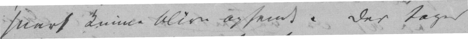snart kunne blive opsendt. Der tages
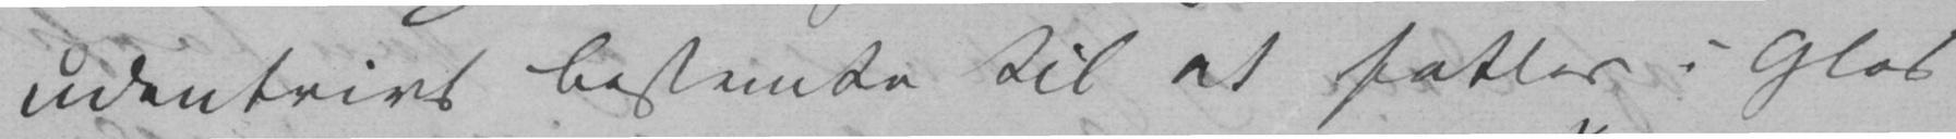udentvivl bestemte til at sættes i Glas
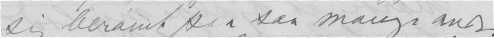sig berømt paa saa mange andres
